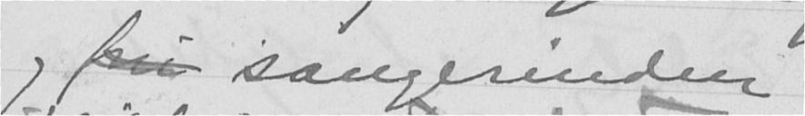og fru sangerinden
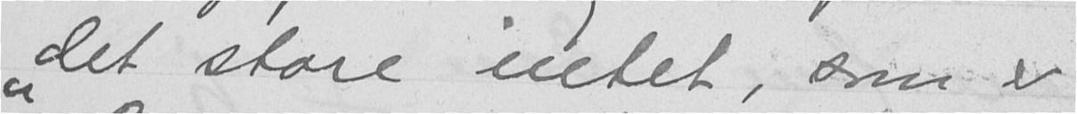"det store intet, som er
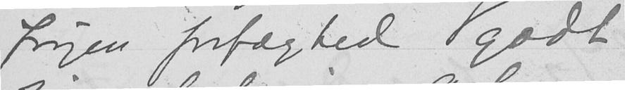Jørgen forfægted godt
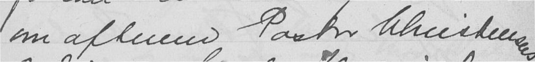om aftenen Pastor Christensens,
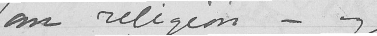om religion - og
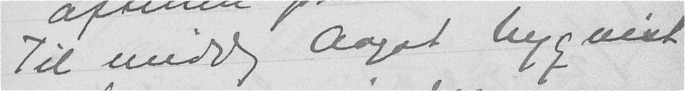Til middag Aagot Nyquist
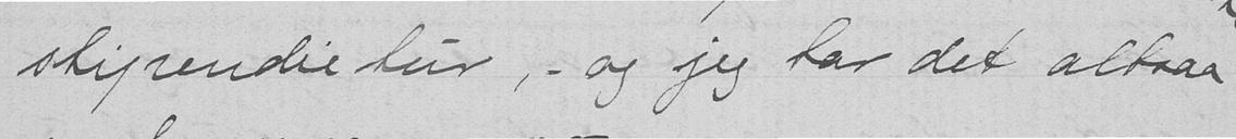stipendietur,- og jeg tar det altsaa
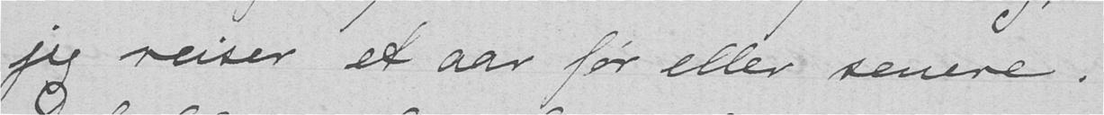jeg reiser et aar før eller senere.
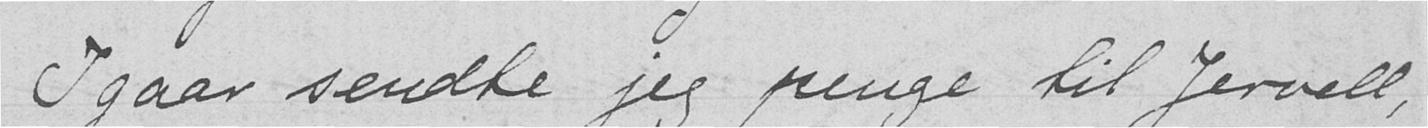Igaar sendte jeg penge til Jervell,
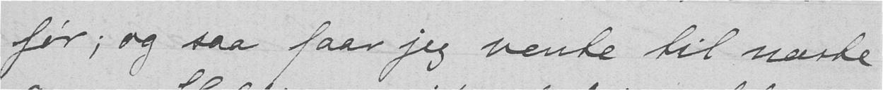før; og saa faar jeg vente til næste
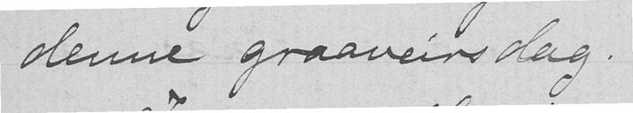denne graaveirsdag.
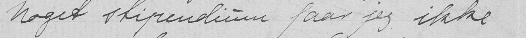Noget stipendium faar jeg ikke
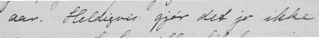aar. Heldigvis gjør det jo ikke
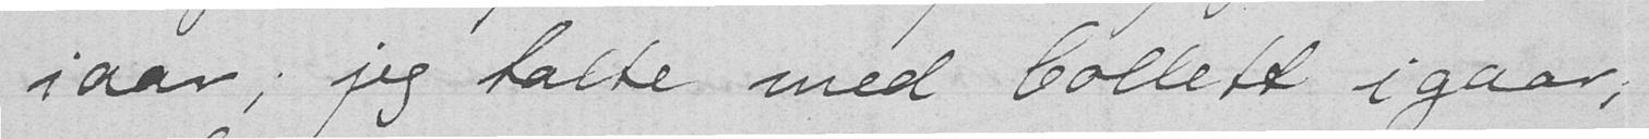i aar; jeg talte med Collett igaar,
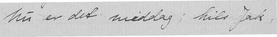Nu er det middag; hils Jak,
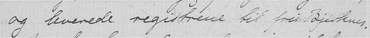og leverede registrene til fru Bjerknes.
